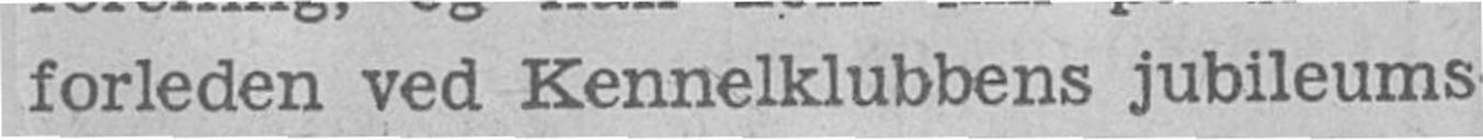forleden ved Kennelklubbens jubileums-
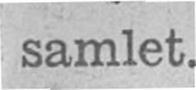samlet.
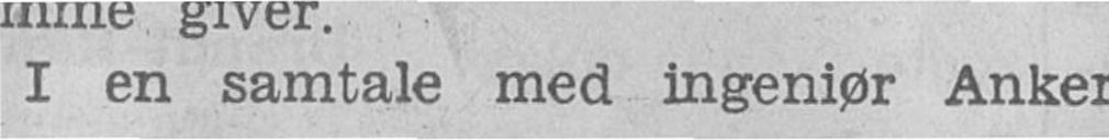I en samtale med ingeniør Anker
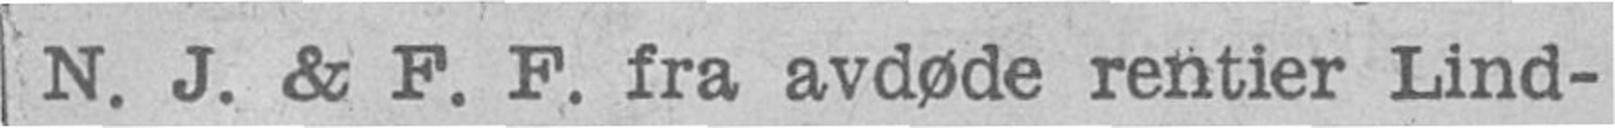N. J. & F. F. fra avdøde rentier Lind¬
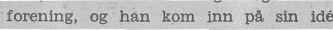forening, og han kom inn på sin idé
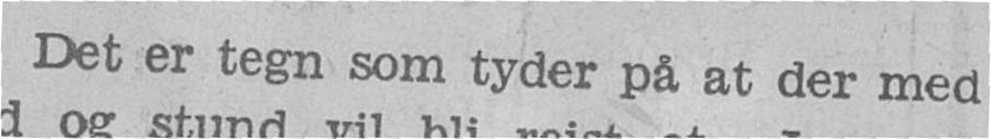Det er tegn som tyder pà at der med
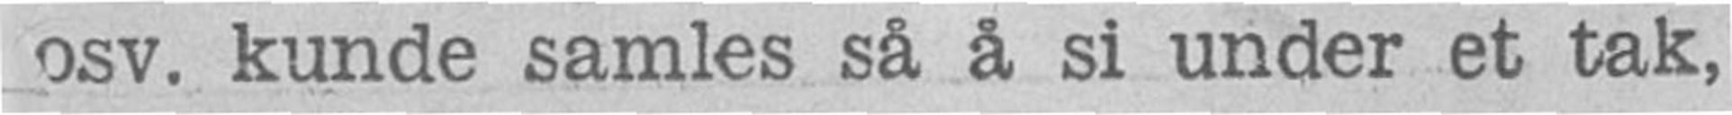osv. kunde samles så å si under et tak,
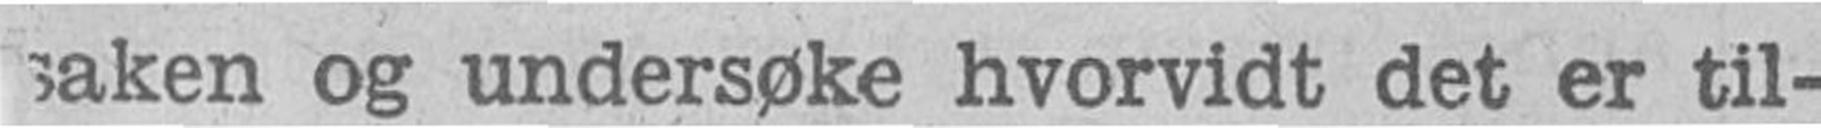saken og undersøke hvorvidt det er til-
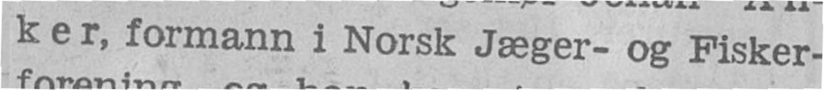ker, formann i Norsk Jæger- og Fisker-
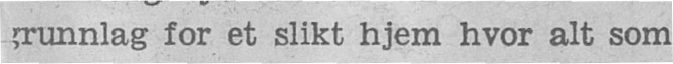grunnlag for et slikt hjem hvor alt som
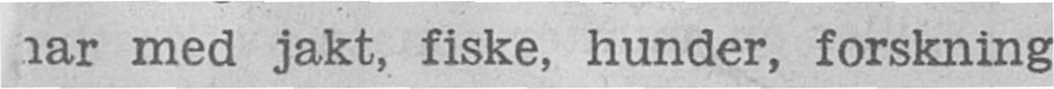ar med jakt, fiske, hunder, forskning
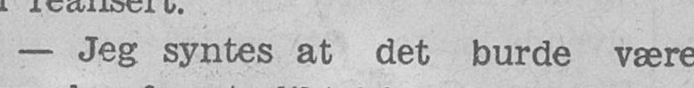- Jeg syntes at det burde være
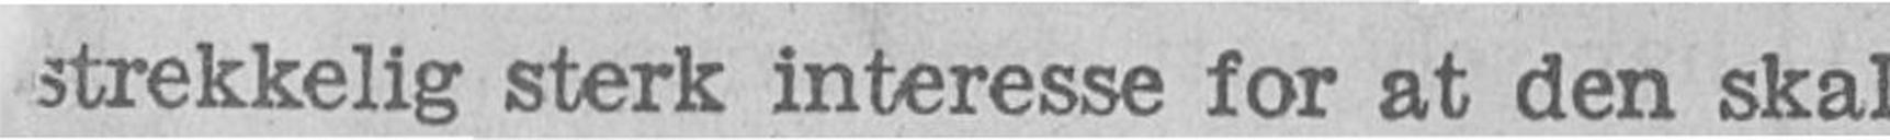strekkelig sterk interesse for at den skal
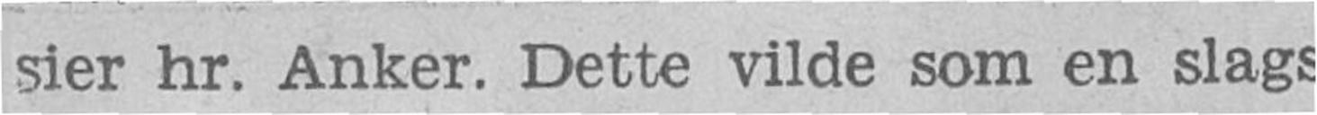sier hr. Anker. Dette vilde som en slags
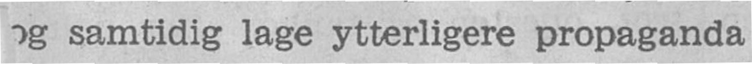og samtidig lage ytterligere propaganda
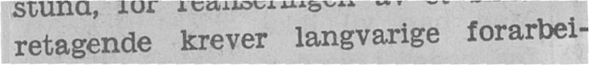retagende krever langvarige forarbei-
